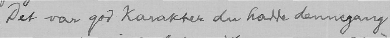Det var god Karakter du hadde dennegang
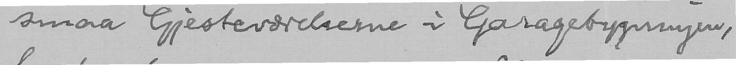smaa Gjesteværelserne i Garagebygningen,
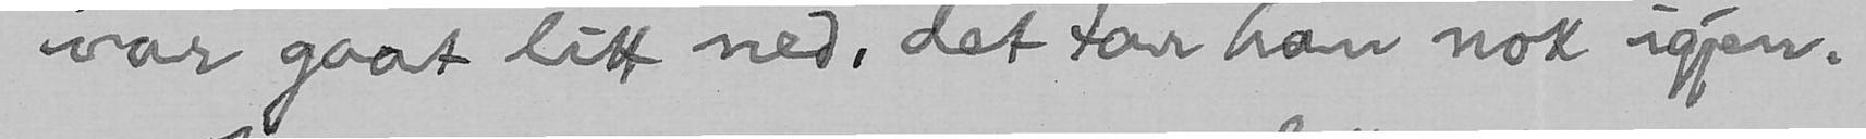var gaat litt ned, det tar han nok igjen.
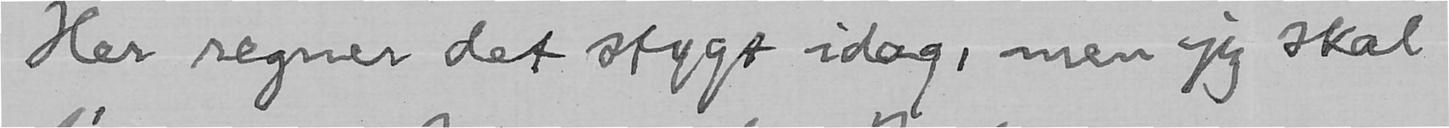Her regner det stygt idag, men jeg skal
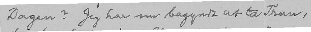Dagen? Jeg har nu begyndt at ta Tran,
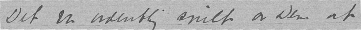Det var ordentlig snilt av Dem at
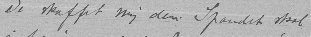De skaffet mig den. Spandet skal
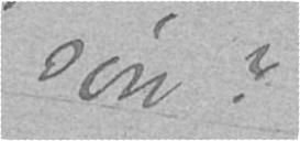søn?
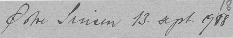Østre Sinsen 13. sept. 1918
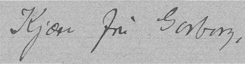Kjære fru Garborg,
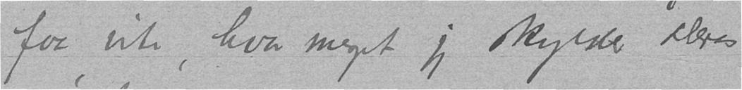faa vite, hvor meget jeg skylder Deres
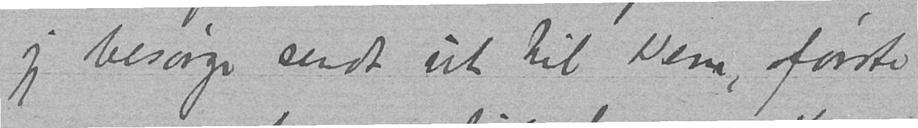jeg besørge sendt ut til Dem, første
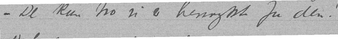– De kan tro vi er henrykte for den!
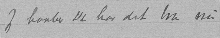Jeg haaber De har det bra nu
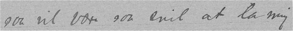saa vil være saa snil at la mig
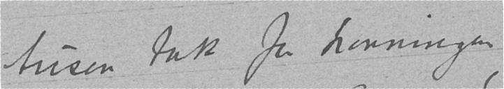tusen tak for honningen
